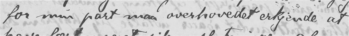for min part maa overhovedet erkjende at
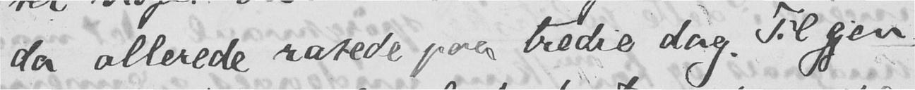da allerede rasede paa tredie dag. Til gjen-
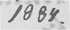1884.
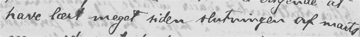have lært meget siden slutningen af marts
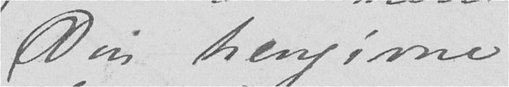Din hengivne
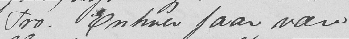Tro. Enhver faar være
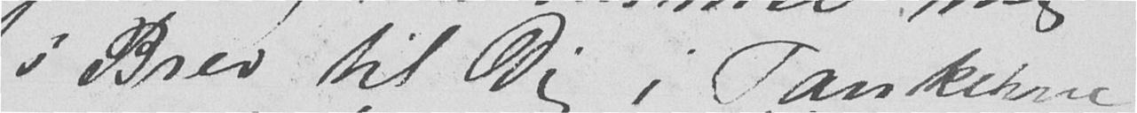i Brev til Dig i Tankerne;
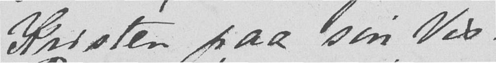Kristen paa sin Vis.
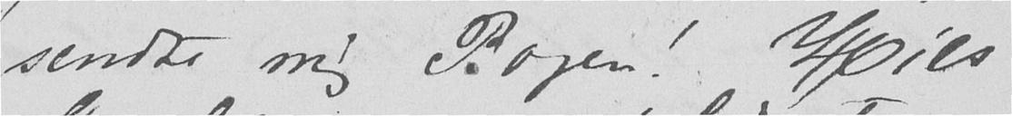sendte mig Bogen! Hils
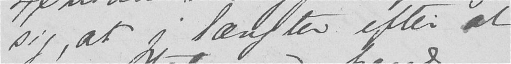sig, at jeg længter efter at
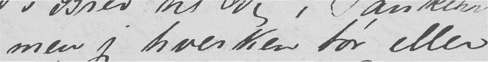men jeg hverken tør eller
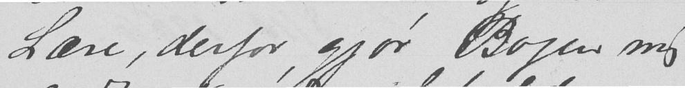Lære, derfor gjør Bogen meg
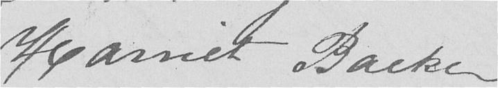Harriet Backer
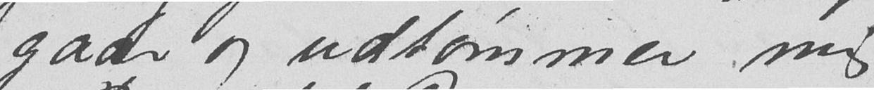gaar og udtømmer mig
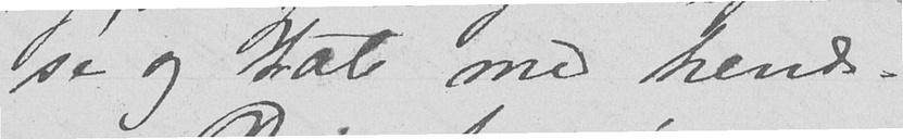se og tale med hende.
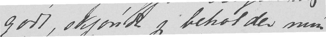godt, skjøndt jeg beholder min
